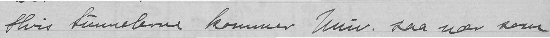Hvis tunnelerne kommer Univ. saa nær som
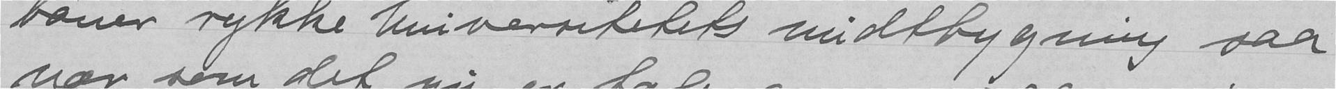baner rykke Universitetets midtbygning saa
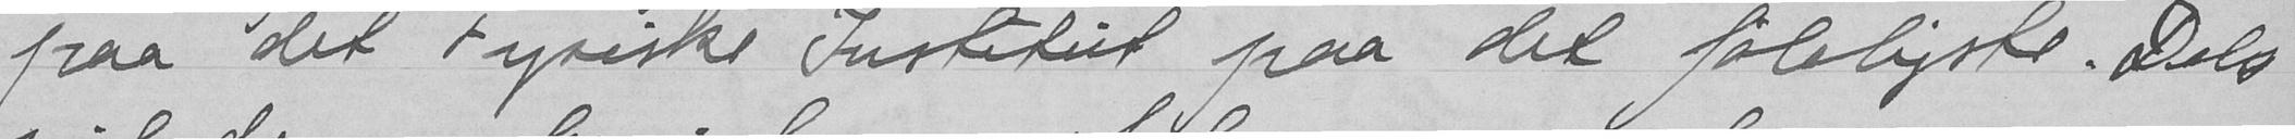paa det Fysiske Institut paa det føleligste. Dels
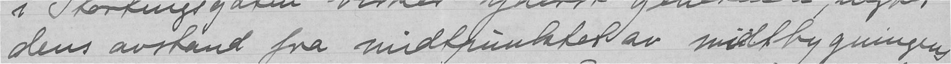dens avstand fra midtpunktet av midtbygningens
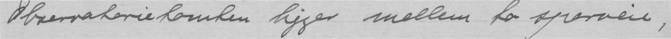Observaterietomten ligger mellem to sporveie,
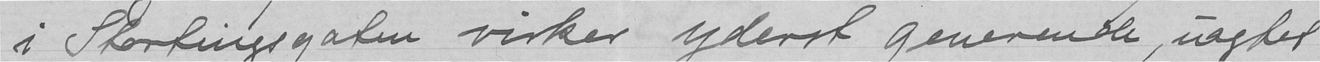i Stortingsgaten virker yderst generende, uagtet
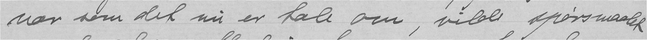nær som det nu er tale om, vilde spørsmaalet
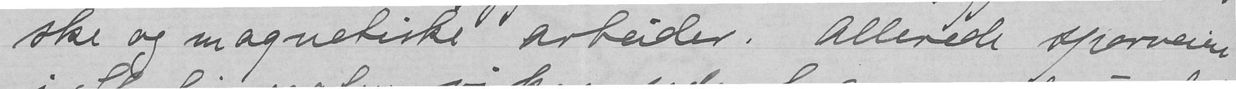ske og magnetiske arbeider. Allerede sporveien
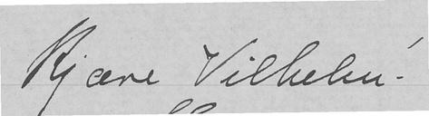Kjære Vilhelm.
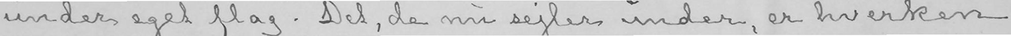under eget flag. Det, de nu sejler under, er hverken
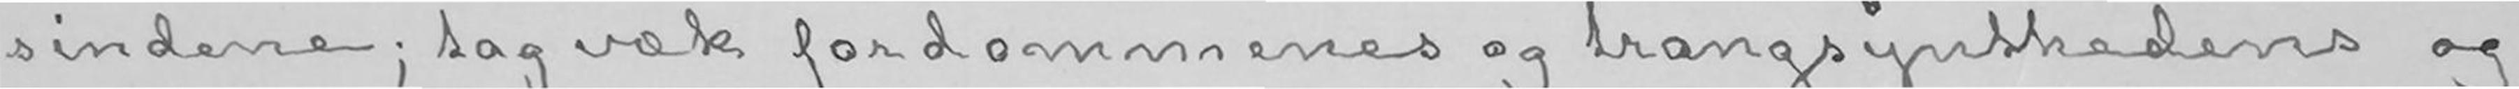sindene; tag væk fordommenes og trangsynthedens og
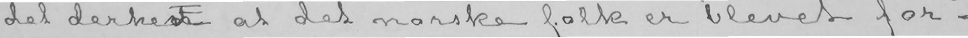det derhen at det norske folk er blevet for-
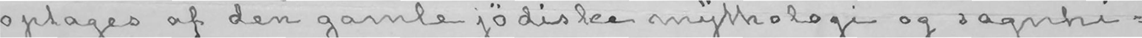optages af den gamle jødiske mythologi og sagnhi-
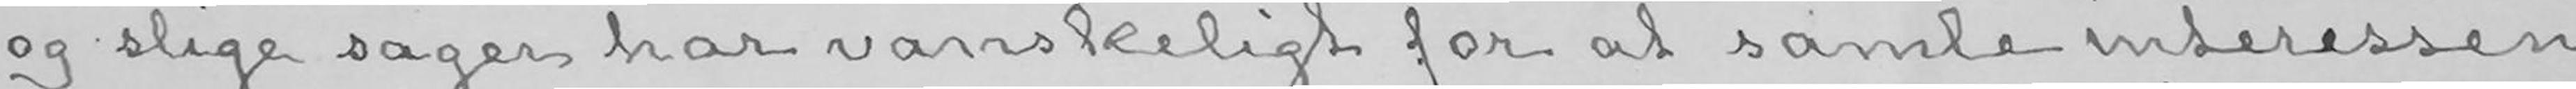og slige sager har vanskeligt for at samle interessen
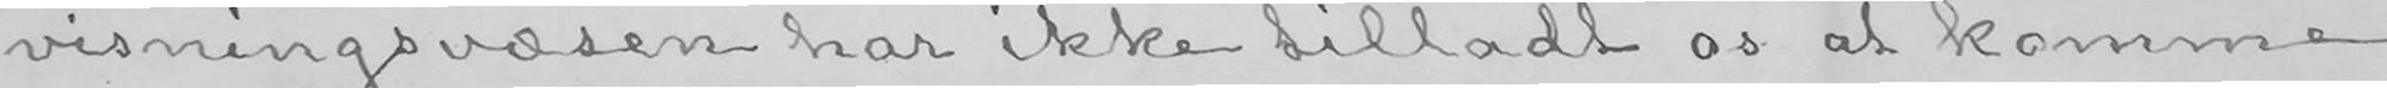visningsvæsen har ikke tilladt os at komme
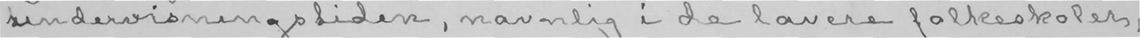undervisningstiden, navnlig i de lavere folkeskoler,
